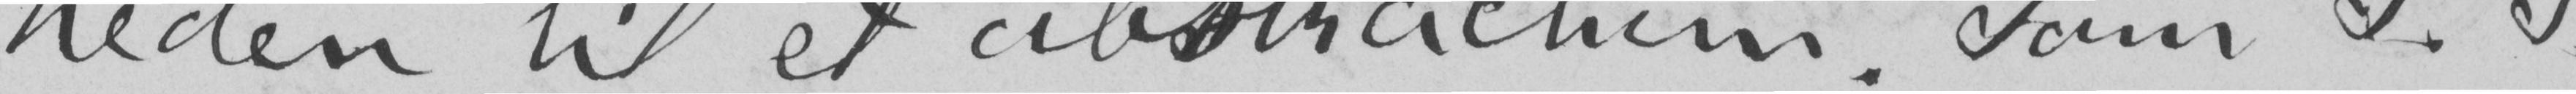heden til et abstractum. Som S.S.
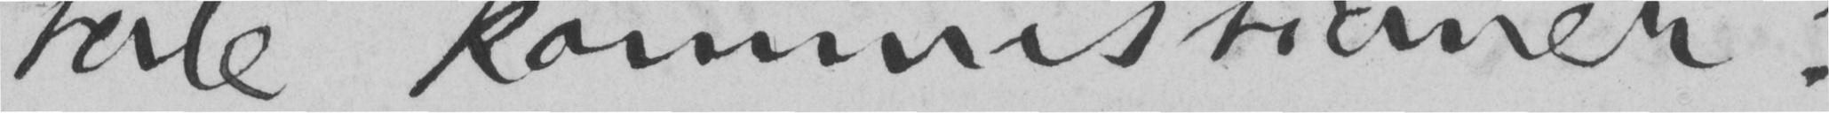tale kommissioner!
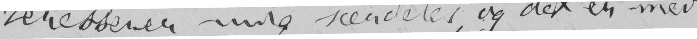teresserer mig særdeles, og det er med
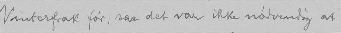Vinterfrak før, saa det var ikke nødvendig at
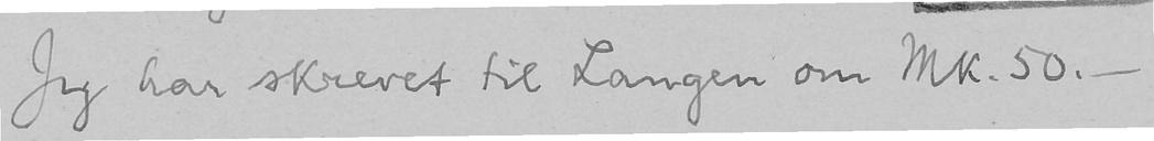Jeg har skrevet til Langen om Mk. 50. -
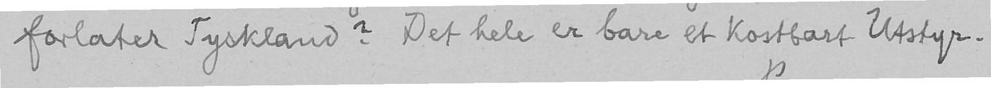forlater Tyskland? Det hele er bare et kostbart Utstyr.
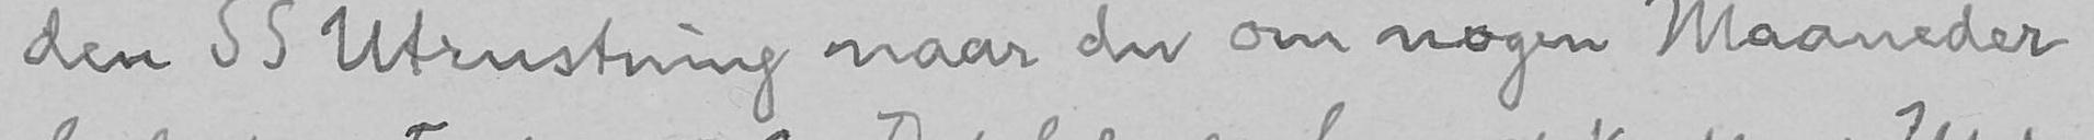den SS Utrustning naar du om nogen Maaneder
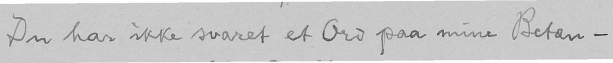Du har ikke svaret et Ord paa mine Betæn-
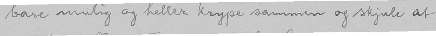bare mulig og heller krype sammen og skjule at
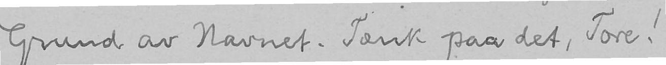Grund av Navnet. Tænk paa det, Tore!
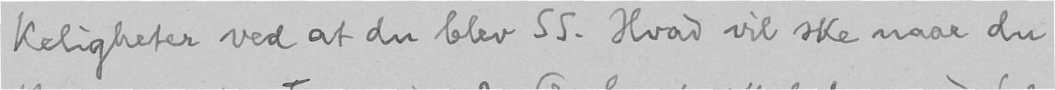keligheter ved at du blev SS. Hvad vil ske naar du
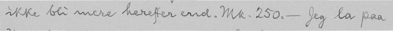ikke bli mere herefter end Mk. 250. - Jeg la paa
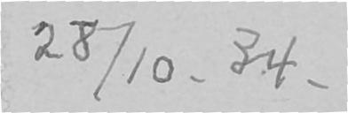28/10. 34.
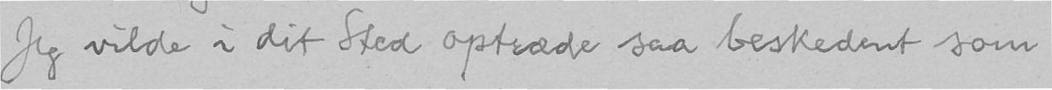Jeg vilde i dit Sted optræde saa beskedent som
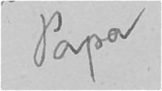Papa
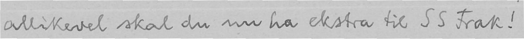allikevel skal du nu ha ekstra til SS Frak!
